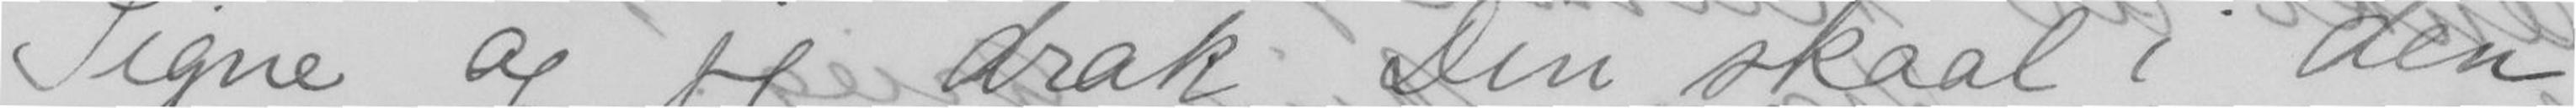Signe og jeg drak Din skaal i den
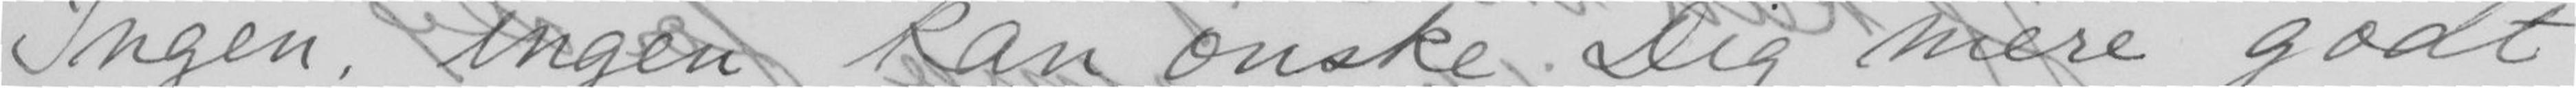Ingen, ingen kan ønske Dig mere godt
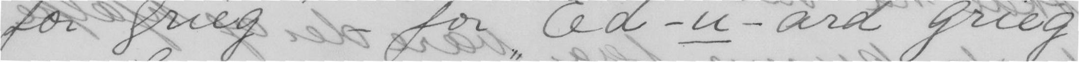for Grieg - for Ed -u- ard Grieg!
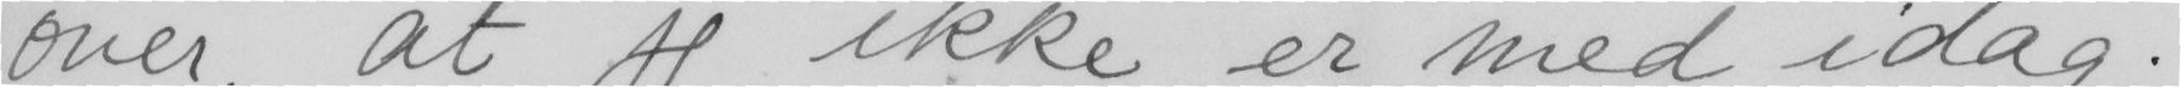over, at jeg ikke er med idag.
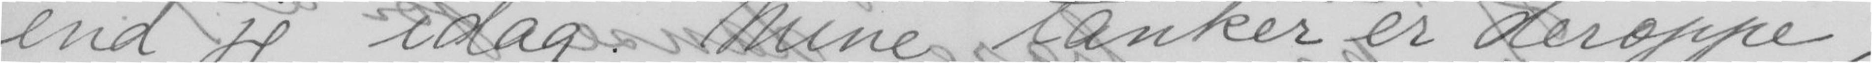end jeg idag. Mine tanker er deroppe,
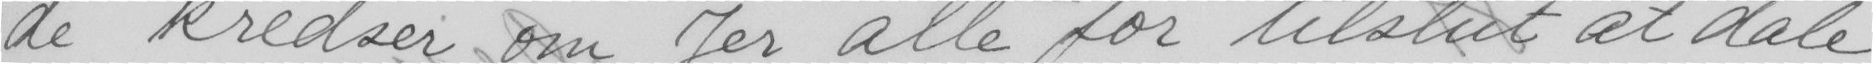de kredser om Jer alle for tilslut at dale
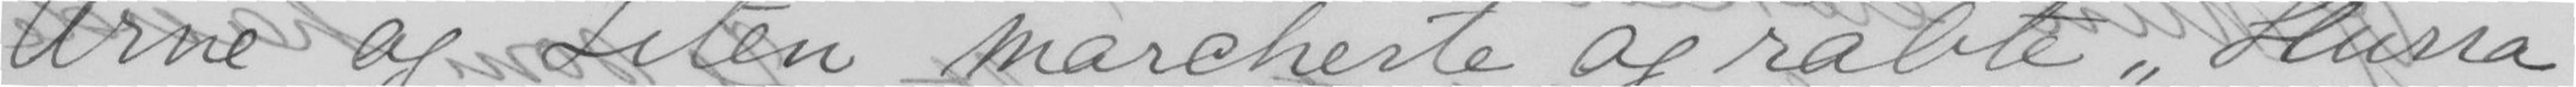Arne og Liten Marcherte og råbte Hurra
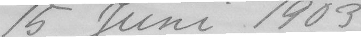15 Juni 1903
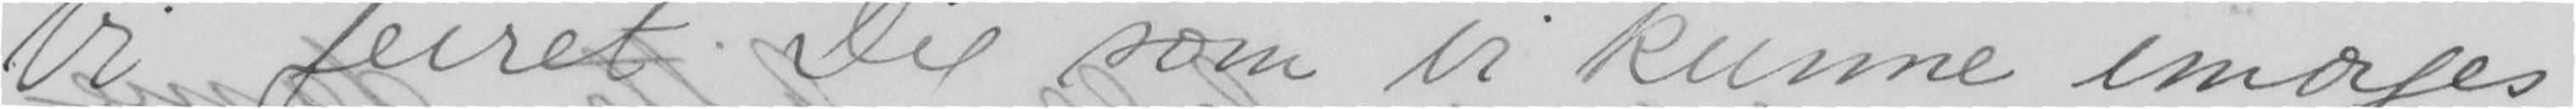Vi feiret Dig som vi kunne imorges,
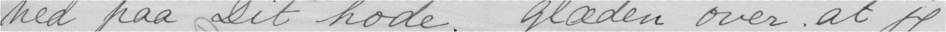ned paa Dit hode. Glæden over at jeg
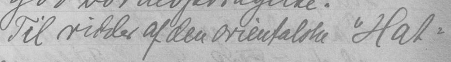Til ridder af den orientalske "Hat-
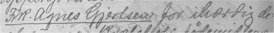Frk. Agnes Gjertsen for ihærdig de-
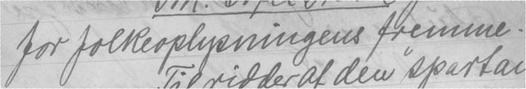for folkeoplysningens fremme.
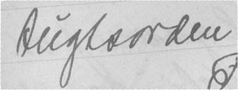tugtsorden"
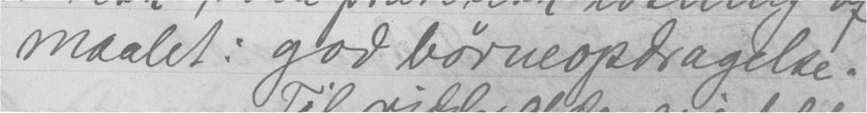maalet: god børneopdragelse.
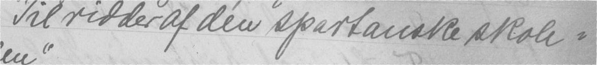Til ridder af den "spartanske skole-
